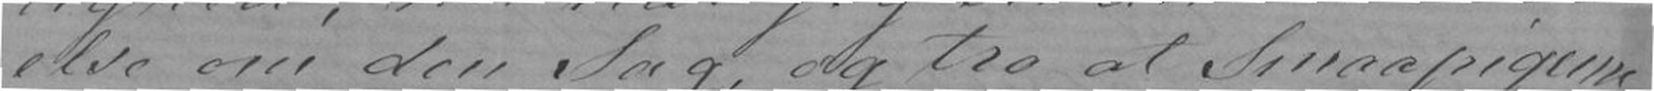else om den Sag, og tro at Smaapigerne
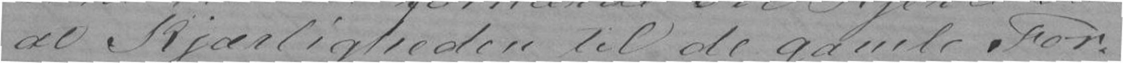at Kjærligheden til de gamle For-
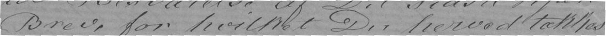Brev, for hvilket Du herved takkes
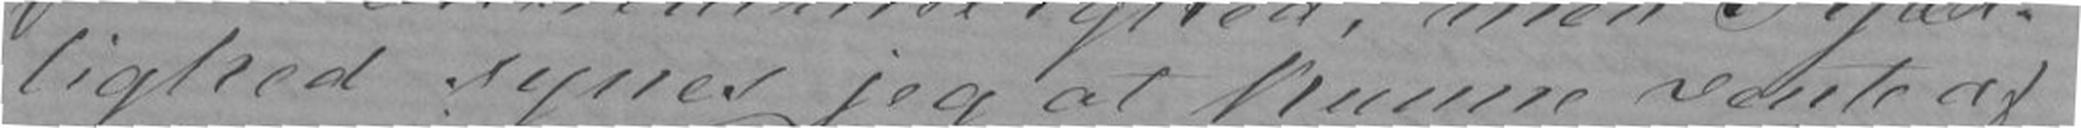lighed synes jeg at kunne vente af
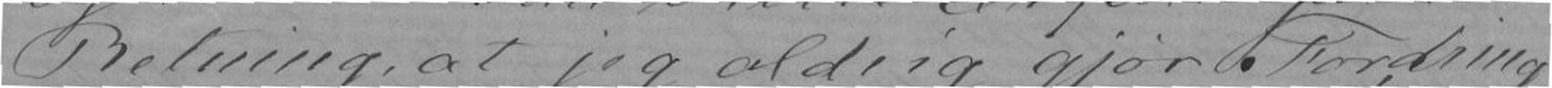Retning, at jeg aldrig gjør Fordring
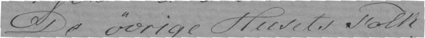De øvrige Husets Folk
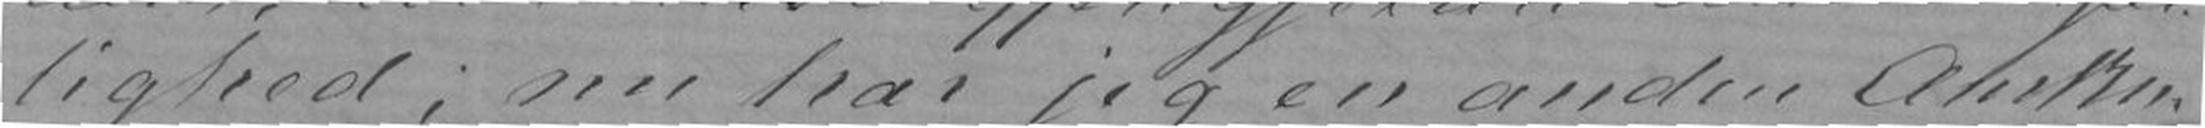lighed; nu har jeg en anden Ansku.
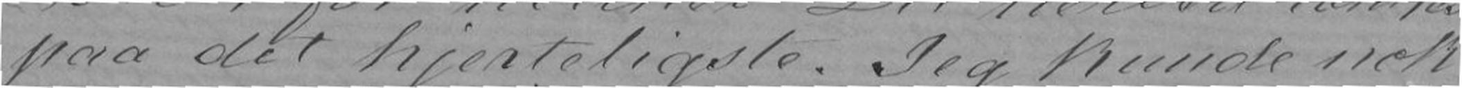paa det hjerteligste. Jeg kunde nok
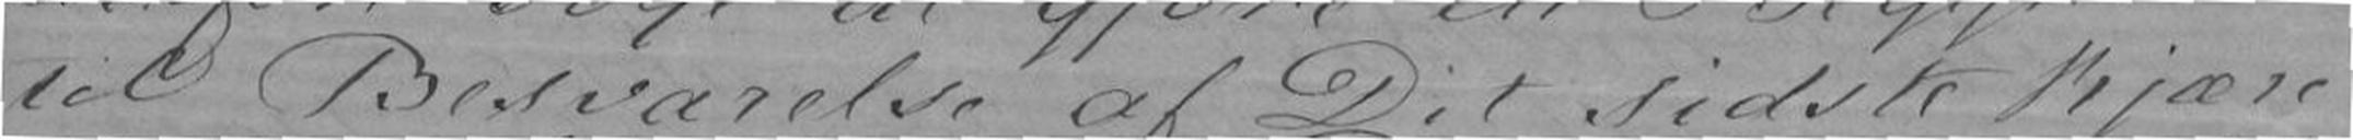til Besvarelse af Dit sidste kjære
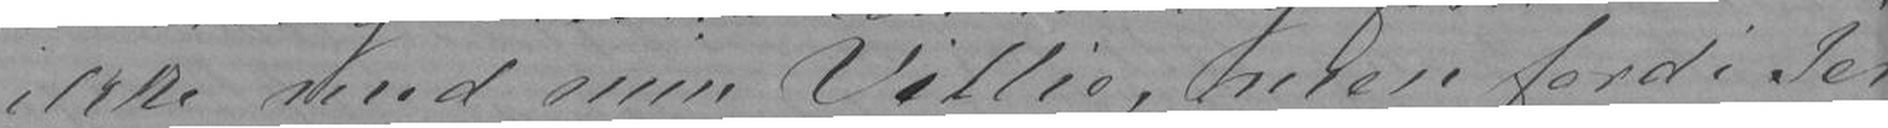ikke med min Villie, men fordi Jer
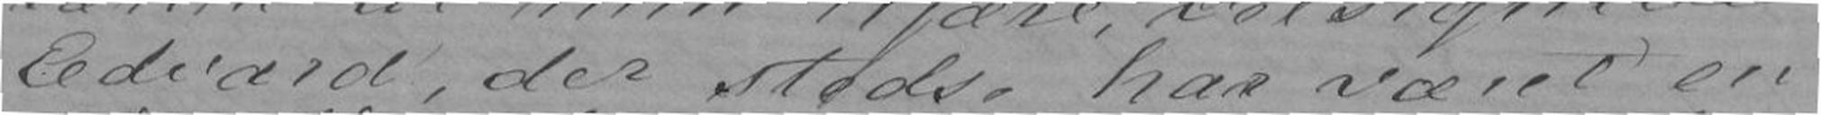Edvard, der stedse har været en
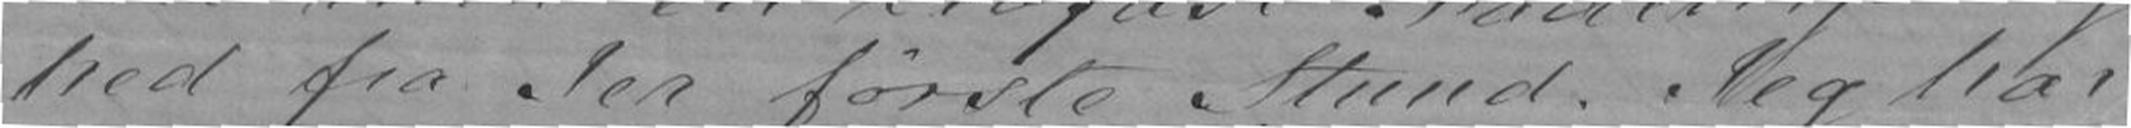hed fra Jer første Stund. Jeg har
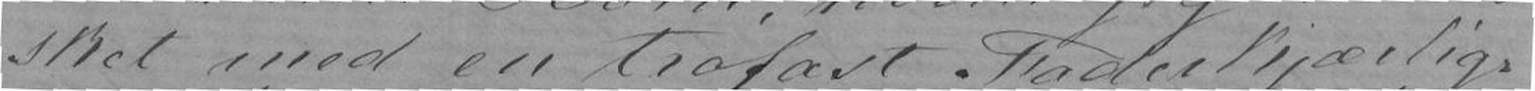sket med en trofast Faderkjærlig-
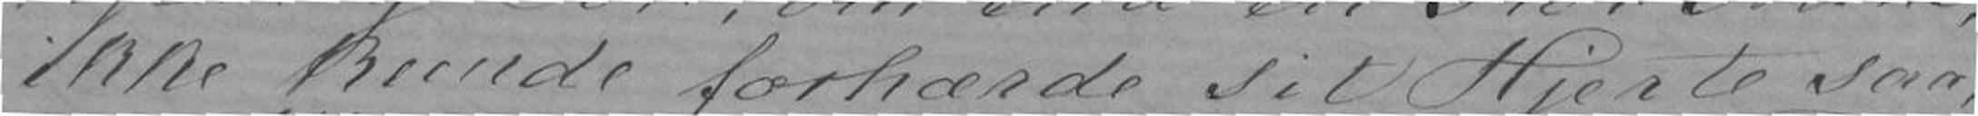ikke kunde forhærde sit Hjerte saa,
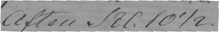Aften Kl.10½
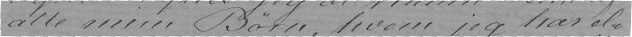alle mine Børn, hvem jeg har el-
